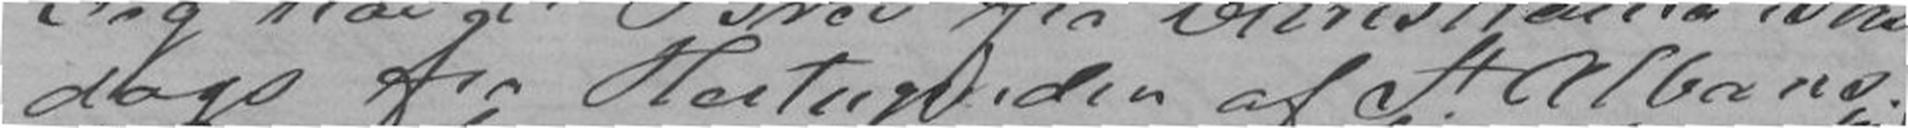dags fra Hertuginden af St. Albans.
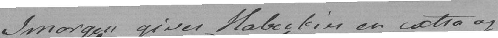Imorgen giver Haberbier en extra og
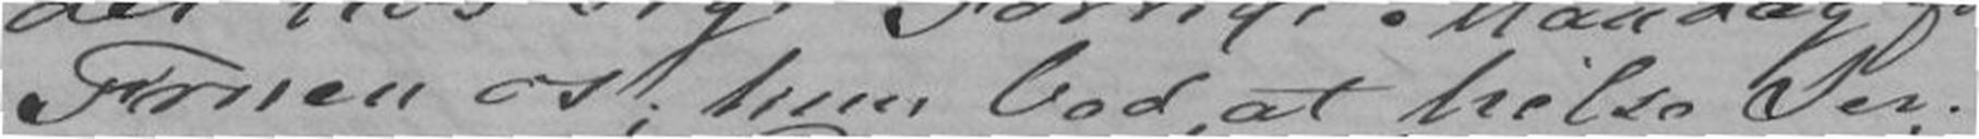Fruen os: hun bad at hilse Jer.
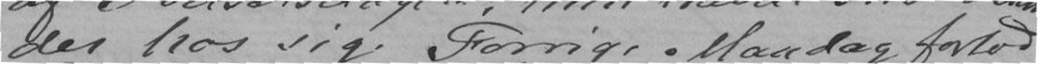der hos sig. Forrige Mandag forlod
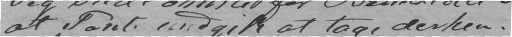at Tante undgik at tage derhen.
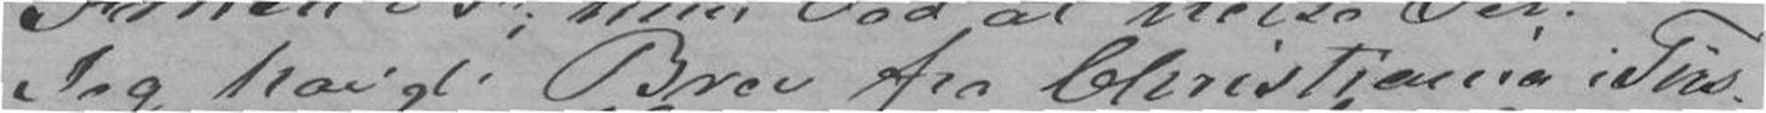Jeg havde Brev fra Christiania i Tirs-
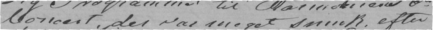Concert, der var meget smuk efter
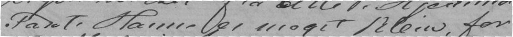Tante Hanne er meget klein, for
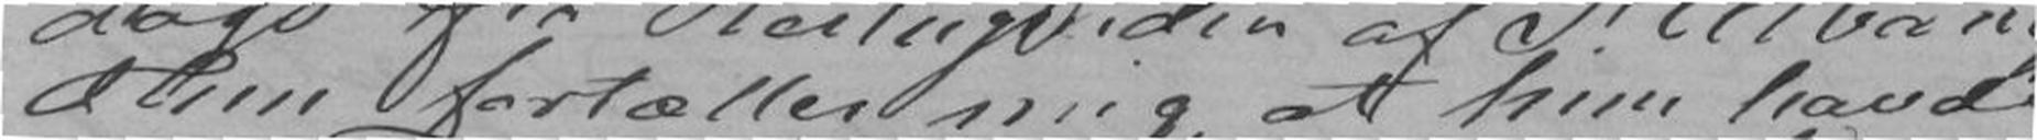Hun fortæller mig at hun havde
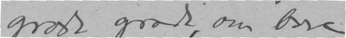græde græde, om bare
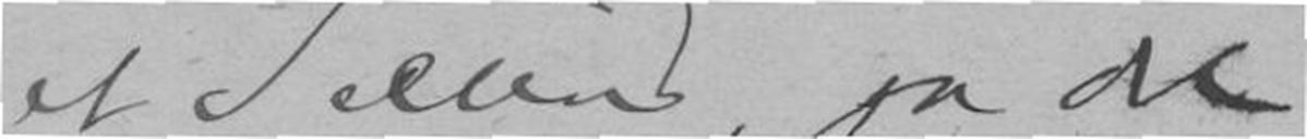et Secund, ja det
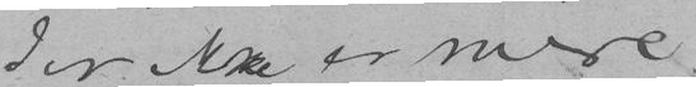der ikke er mere. -
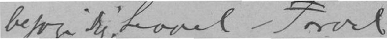besøge dig. Levvel - Farvel
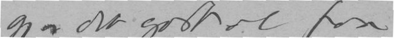gjør det godt at faa
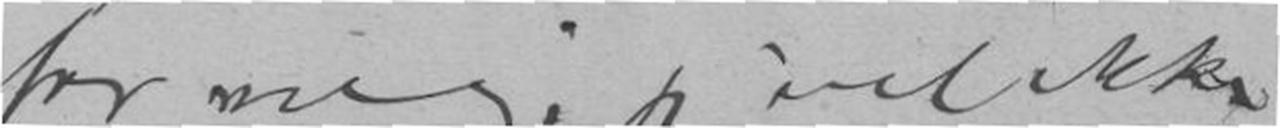for mig, jeg vil ikke
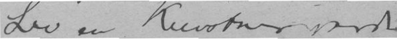Lev en Kunstner værdig
